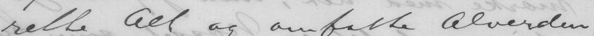rette Alt og omfatte Alverden,
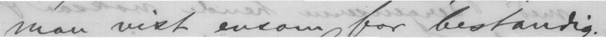man vist ensom for bestandig.
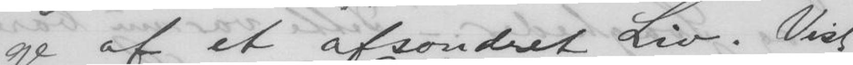ge af et afsondret Liv. Vist
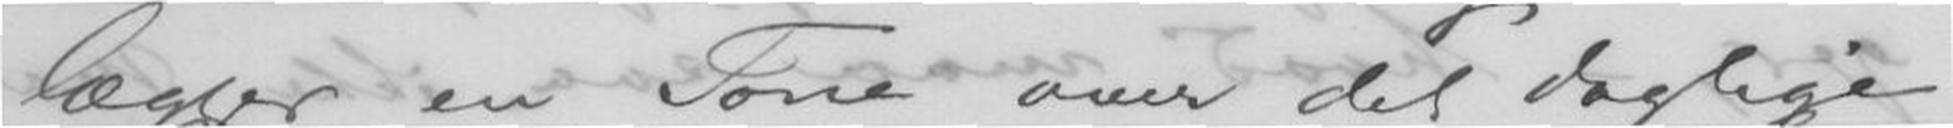lægger en Tone over det daglige
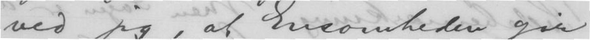ved jeg, at Ensomheden gir
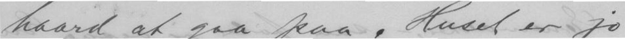haard at gaa paa. Huset er jo
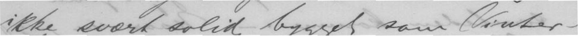ikke svært solid bygget som Vinter-
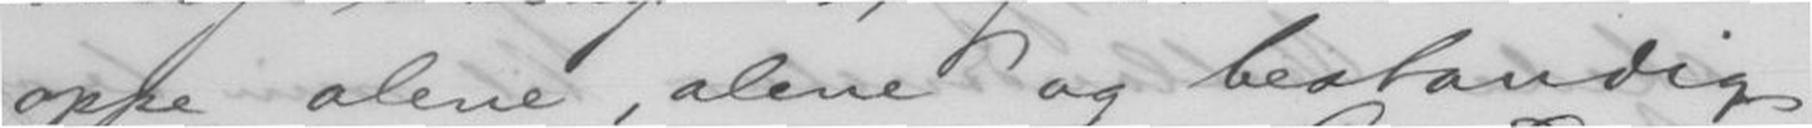oppe alene, alene og bestandig
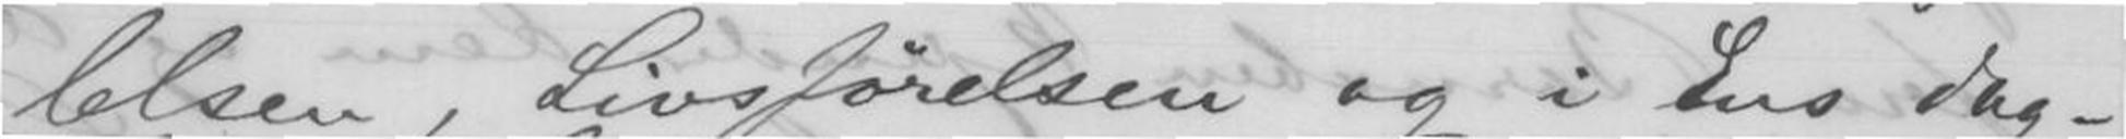lelsen, Livsførelsen og i Ens dag-
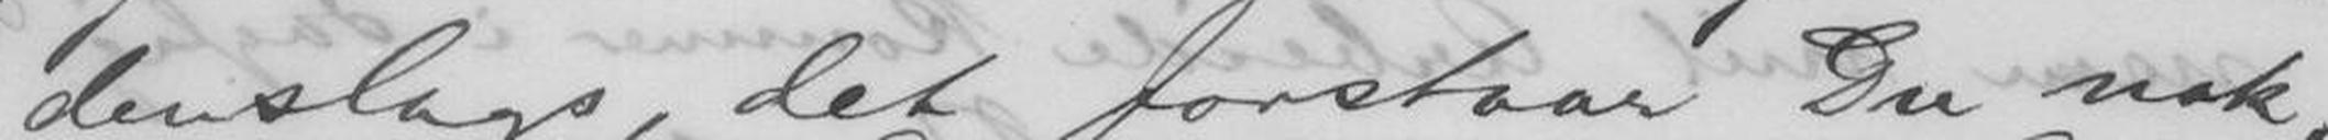denslags, det forstaar Du nok,
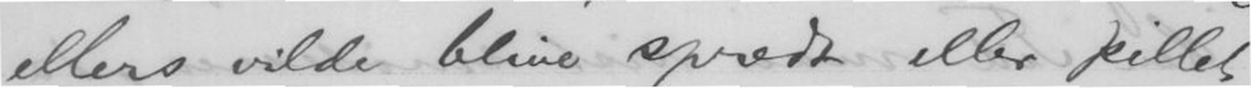ellers vilde blive spredt eller pillet
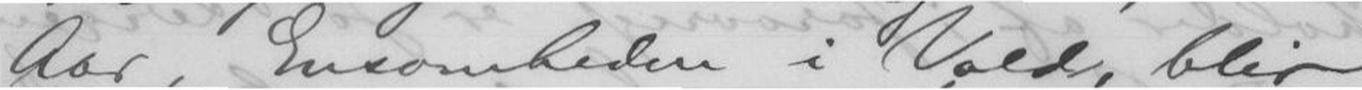Aar, Ensomheden i Vold, blir
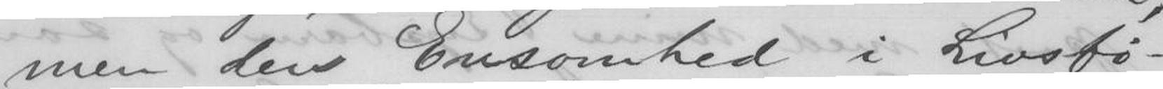men den Ensomhed i Livsfø-
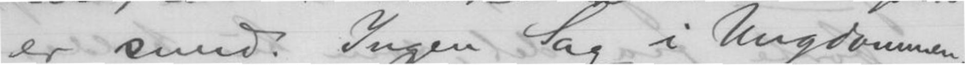er sund. Ingen Sag i Ungdommen,
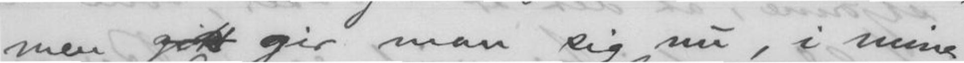men gitt gir man sig nu, i mine
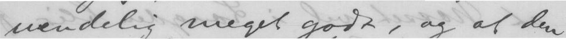uendelig meget godt, og at den
